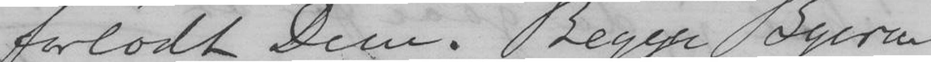forlodt Dem. Begge Byerne
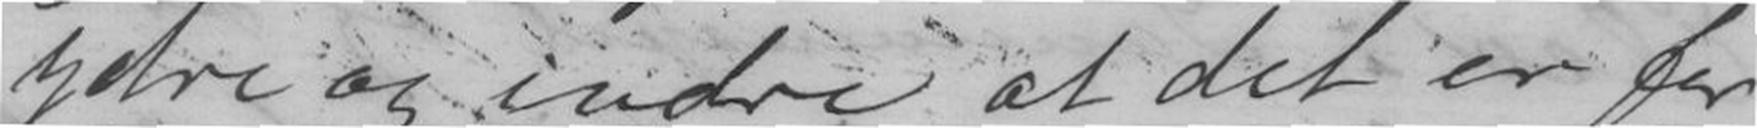ydre og indre at det er for
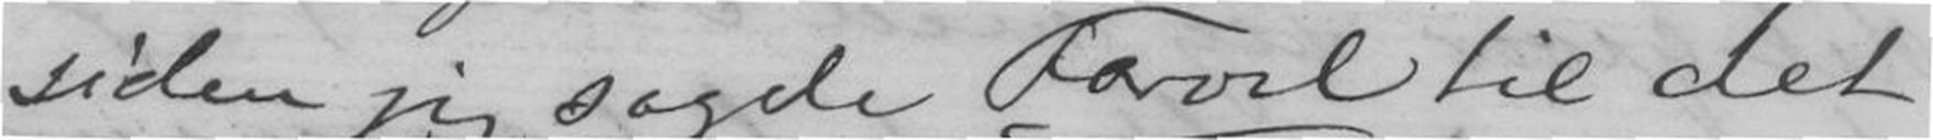siden jeg sagde Farvel til det
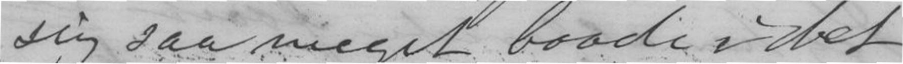sig saa meget baade i det
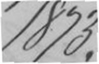1873.
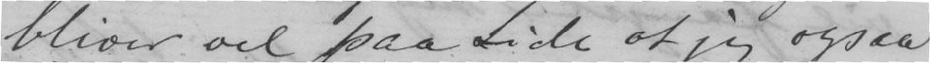bliver vel paa tide at jeg ogsaa
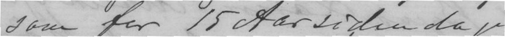som for 15 Aar siden da jeg
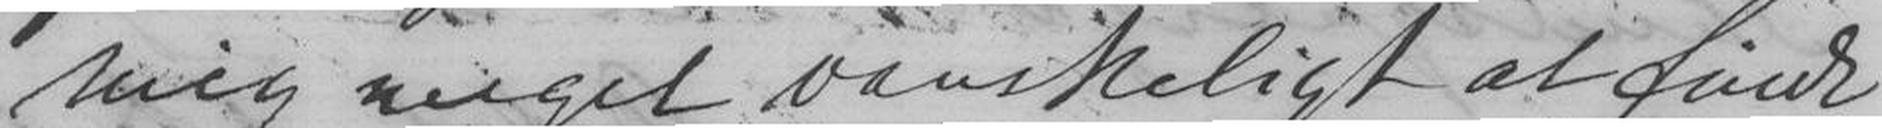mig meget vanskeligt at finde
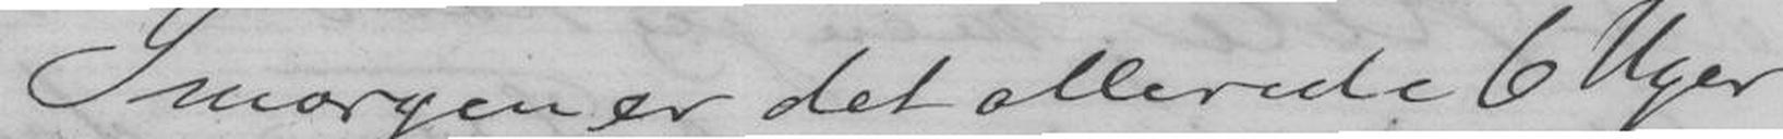Imorgen det allerede 6 Uger
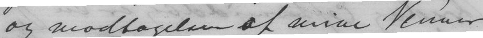og modtagelsen af mine Venner
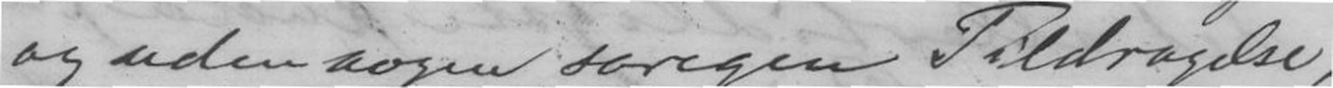og uden nogen soregen Tildragelse,
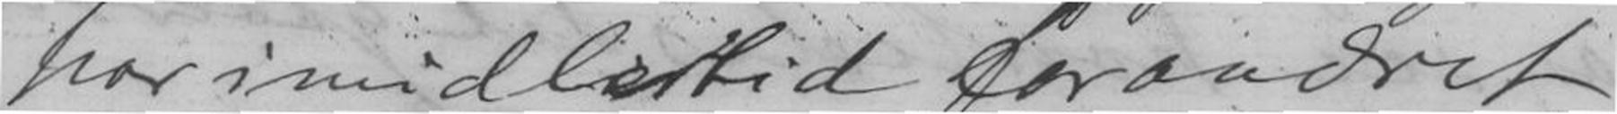har imidlertid forandret
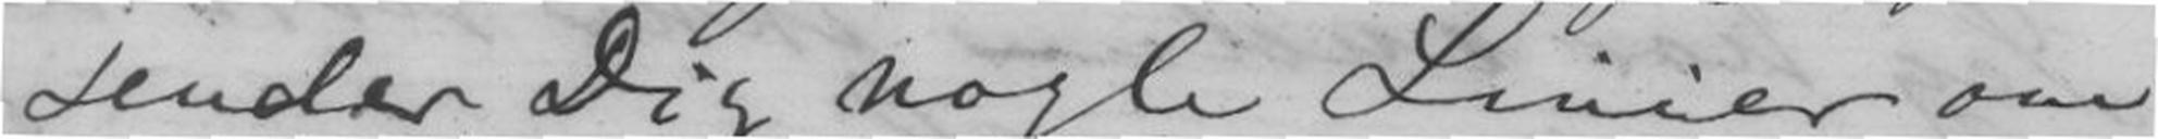sender Dig nogle Linier om
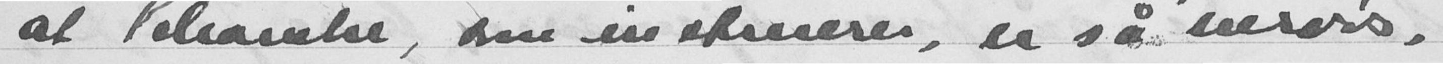at Schanke, som instruerer. er så nervøs,
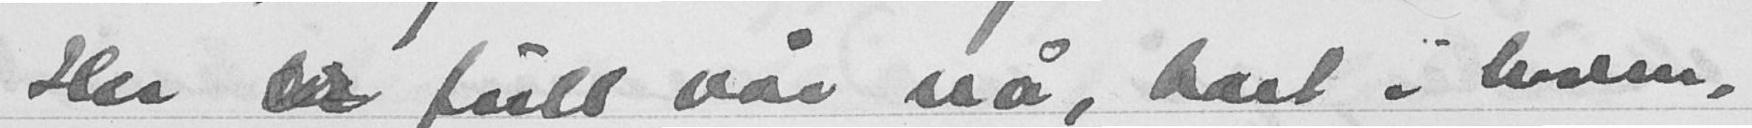Her er full vår nå, bart i haven,
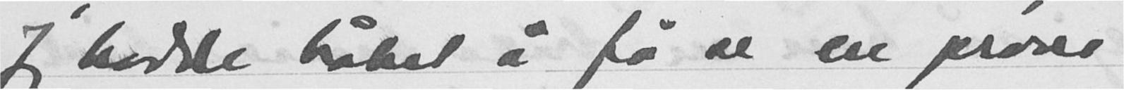Jeg hadde håbet i få av en prøve
